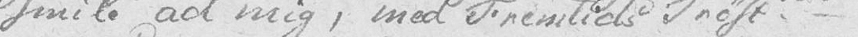smile ad mig, med Fremtids Trøst. –
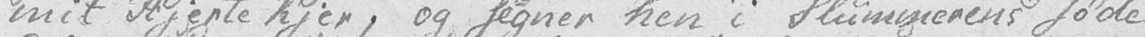mit Hjerte kjer, og segner hen i Slummerens søde
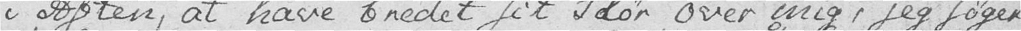i Aften, at have bredet sit Slør over mig, jeg søger
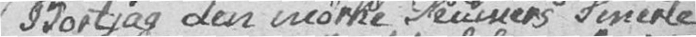Bortjag den mørke Kummers Smerte
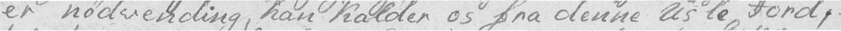er nødvending, han kalder os fra denne Usle Jord, –
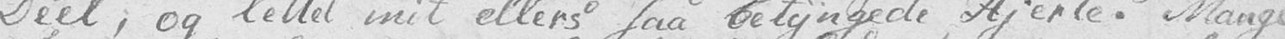Deel, og lettet mit ellers saa betyngede Hjerte. Mange
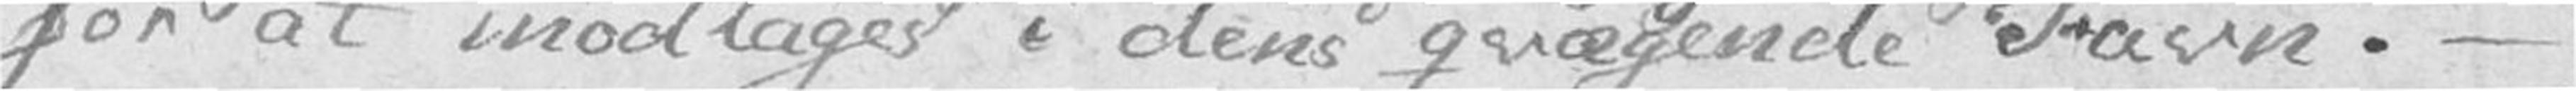for at modtages i dens qvægende Favn. –
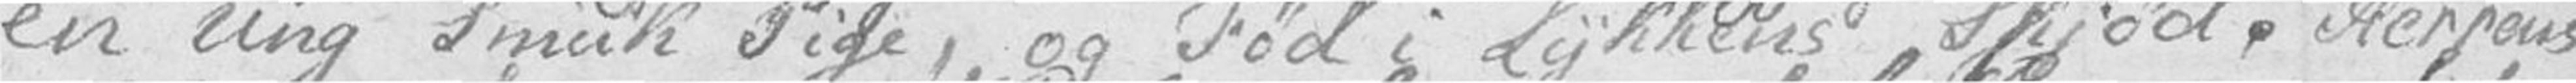en ung Smuk Pige, og Fød i Lykkens Skjød. Herrens
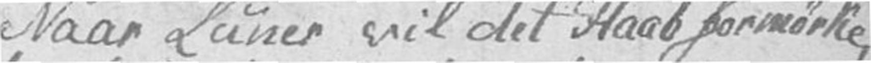Naar Luner vil det Haab formørke,
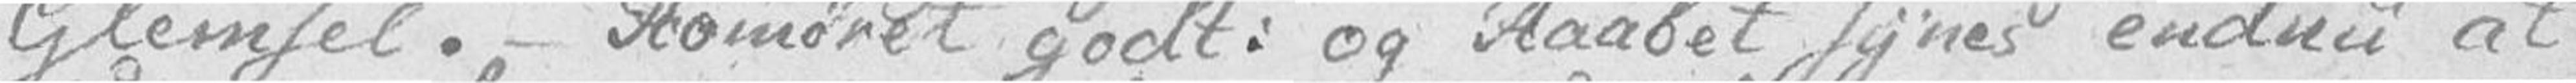Glemsel. – Homøret godt: og Haabet synes endnu at
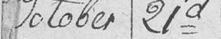October 21d
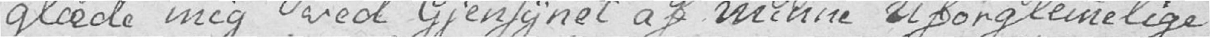glæde mig ved Gjensynet af minne uforglemmelige
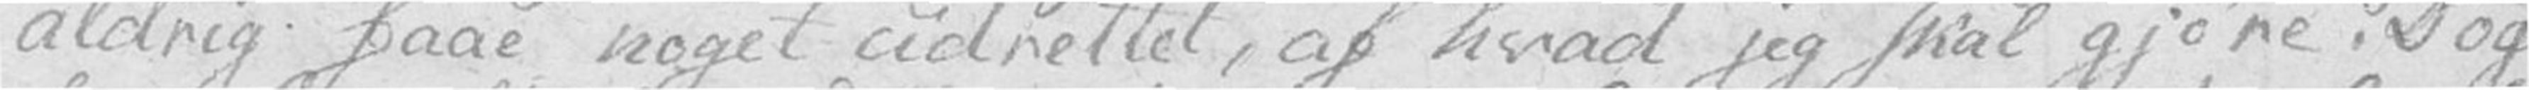aldrig faae noget udrettet, af hvad jeg skal gjøre. Dog
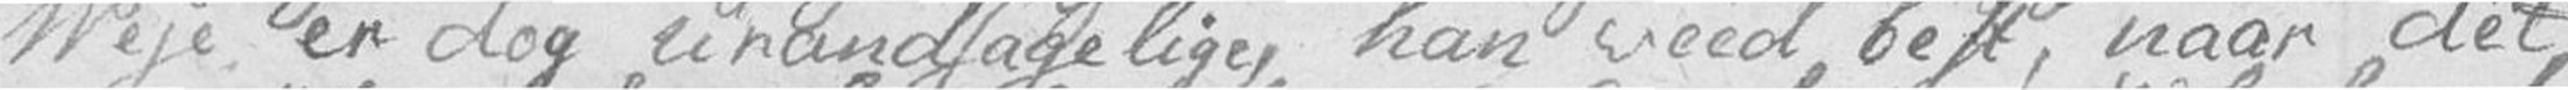Veje er dog urandsagelige, han veed best, naar det
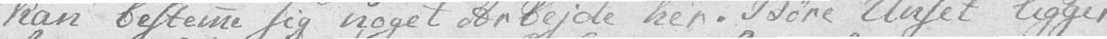kan bestemme sig noget Arbejde her. Børe Unset ligger
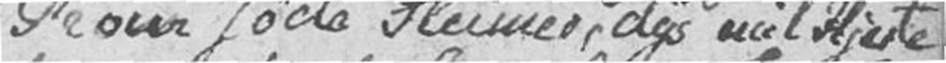Kom søde Slummer, dys mit Hjerte
